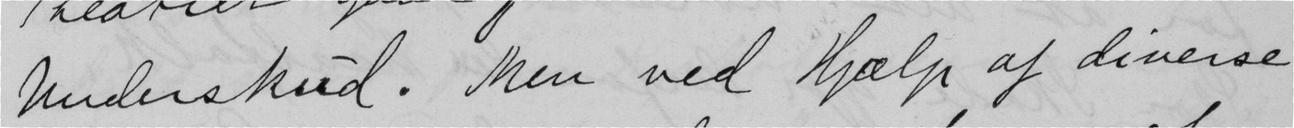Underskud. Men ved Hjælp af diverse
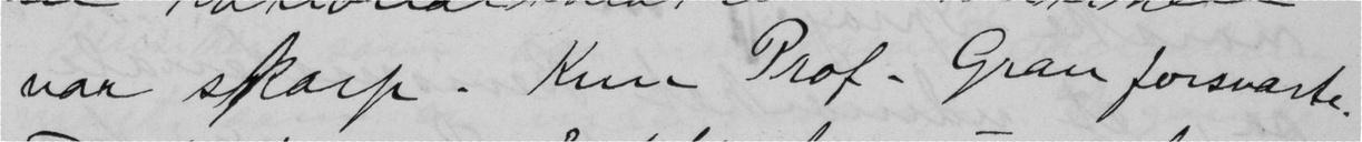var skarp. Kun Prof. Gran forsvarte.
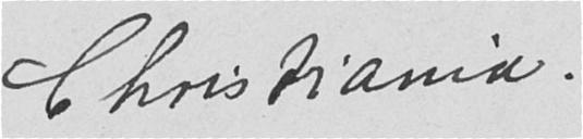Christiania.
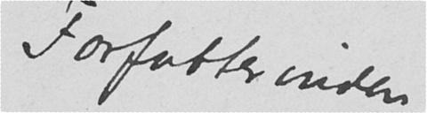Forfatterinden
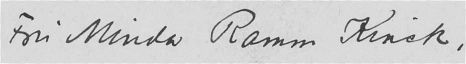Fru Minda Ramm Kinck,
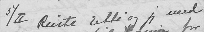5/II Reiste Etti og jeg med
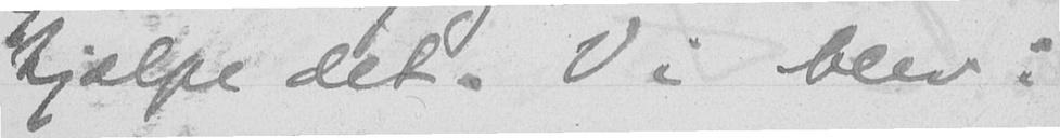hjælpe det. Vi blev i
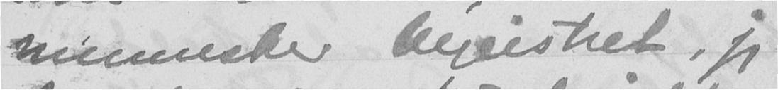mennesker begeistret, jeg
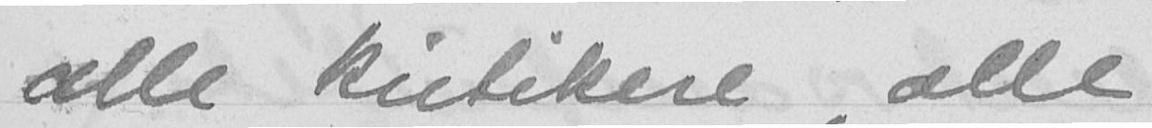alle kritikere, alle
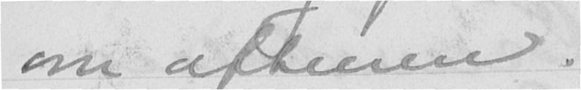om aftenen.
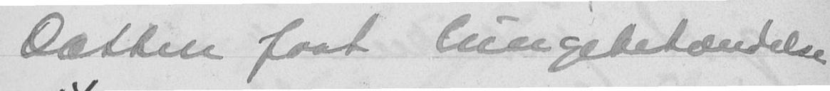Dotten faat lungebetændelse.
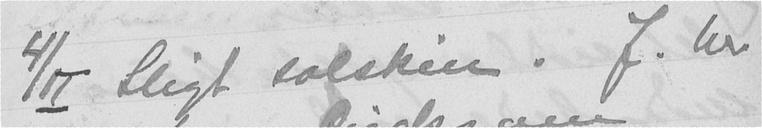4/II Sligt solskin. J. her
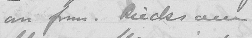om form. Riecks om
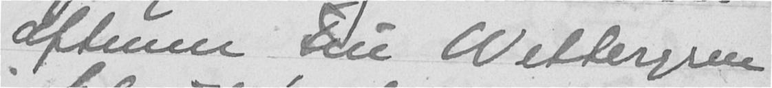aftenen Fru Wettergren
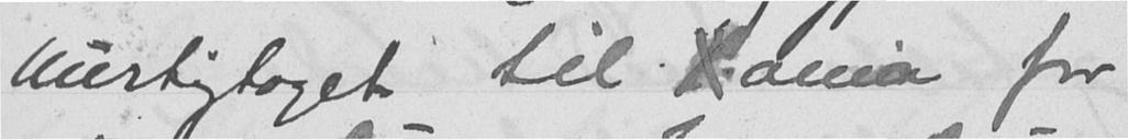hurtigtoget til Xania for
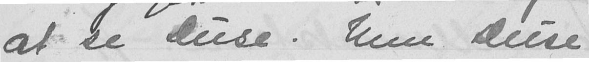at se Duse. Men Duse
